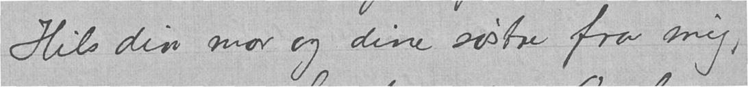Hils din mor og dine søstre fra mig,
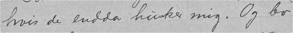hvis de endda husker mig. Og lev
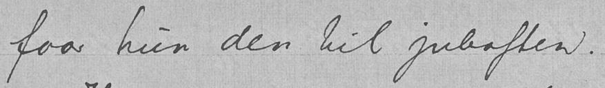faar hun den til julaften.
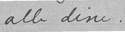alle dine.
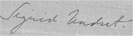Sigrid Undset.
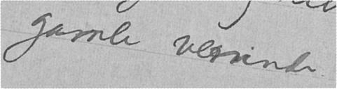gamle veninde
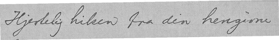Hjertelig hilsen fra din hengivne
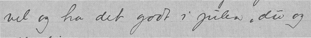vel og ha det godt i julen, du og
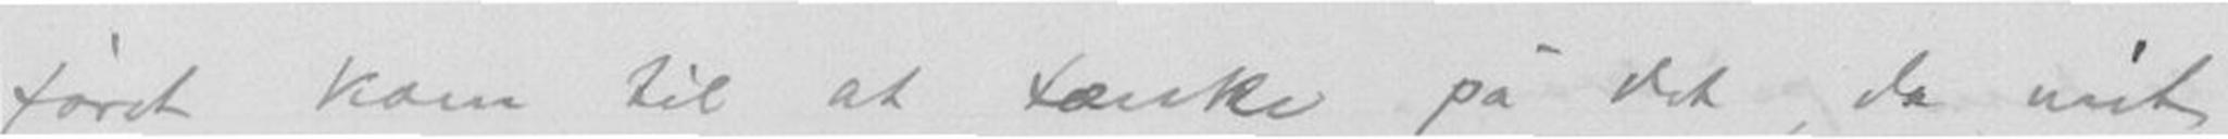først kom til at tænke på det, da mit
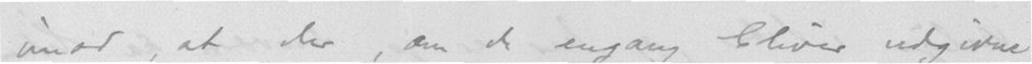imod, at der, om de engang bliver udgivne,
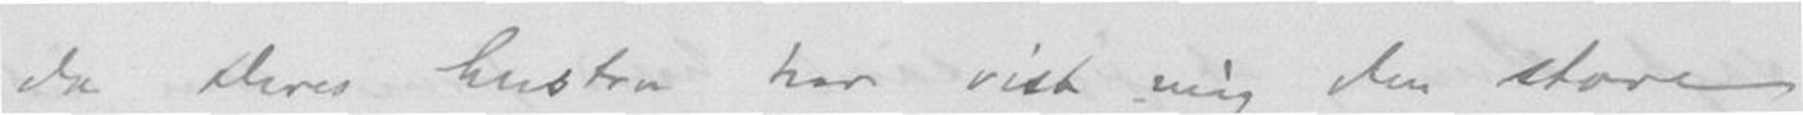da Deres hustru har vist mig den store
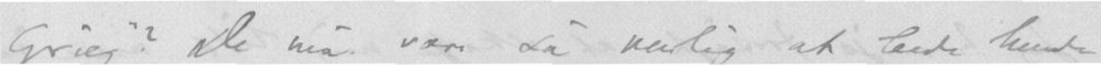Grieg"? De må være så venlig at bede hende
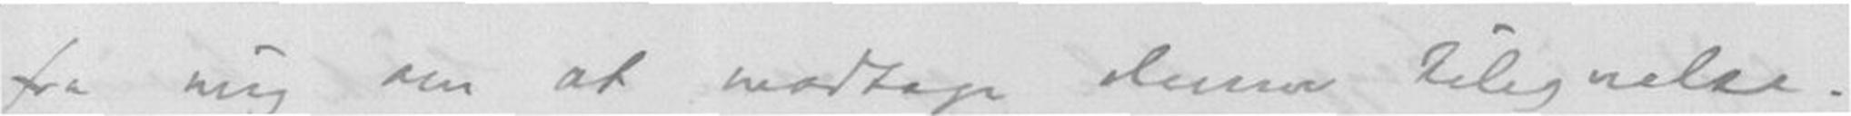fra mig om at modtage denne tilegnelse.
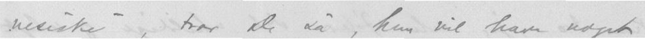nesiske, tror De så, hun vil have noget
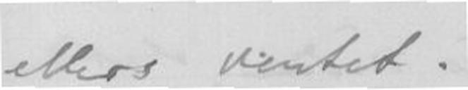ellers ventet.
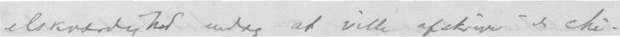elskværdighed endog at ville afskrive de chi-
